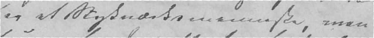er et Stykværksmenneske, men
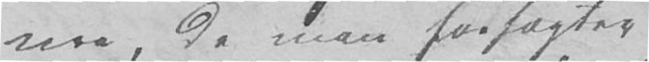me, da man forfegter
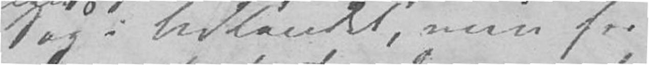Sag i Udlandet, men for
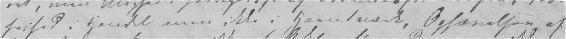frihed i Handel men ikke i Haandværk, Ophævelsen af
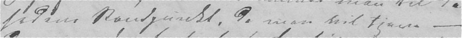hedens Standpunkt, da man vil tjene –
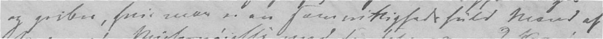og gribes, hvis man er en samvittighedsfuld Mand af
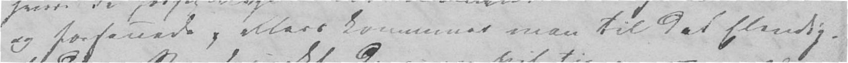og forsonede, ellers kommer man til det Elendig-
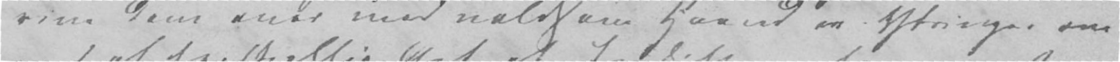rive dem over med voldsom Haand er Ytringer om
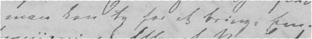man kan ty for at bringe Een-
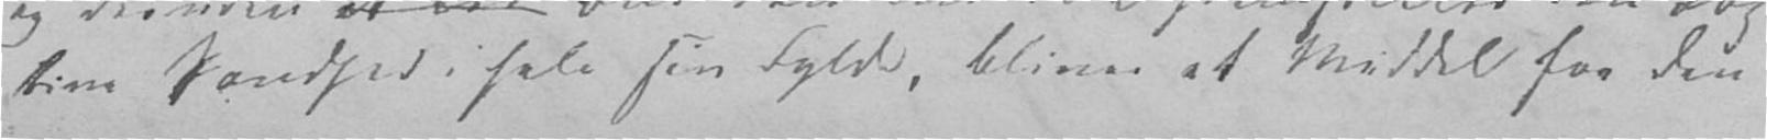tive Sandhed i hele sin Fylde, bliver et Middel for den
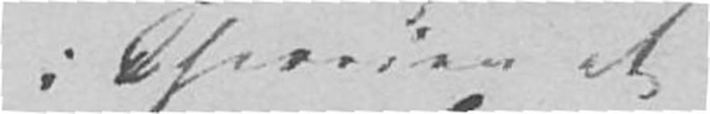i Theorien at
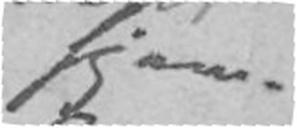Hjem-
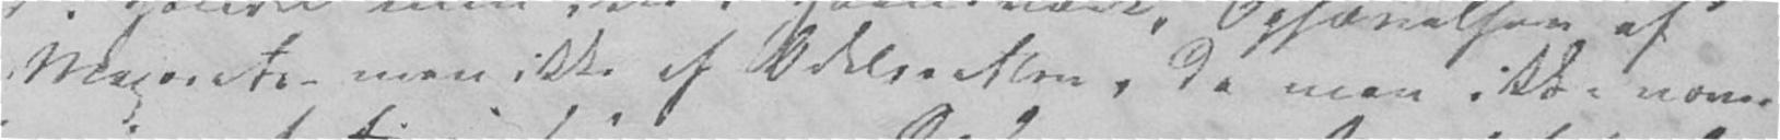Majorats- men ikke af Odelsretten, da man ikke vover
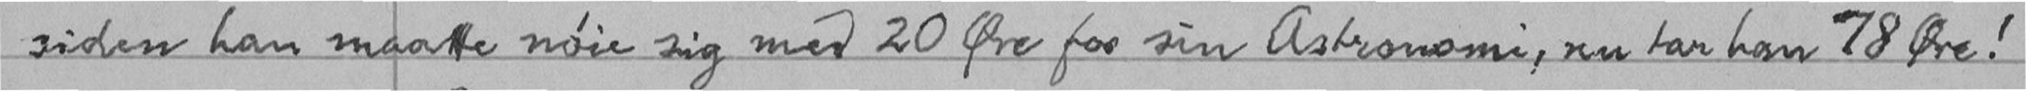siden han maatte nøie sig med 20 Øre for sin Astronomi, nu tar han 78 Øre!
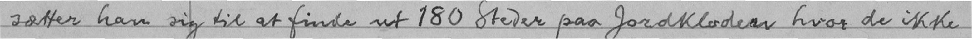sætter han sig til at finde ut 180 Steder paa Jordkloden hvor de ikke
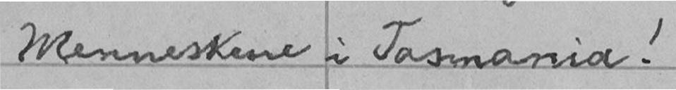Menneskene i Tasmania!
